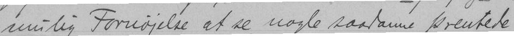mulig Fornøjelse at se nogle saadanne prentede
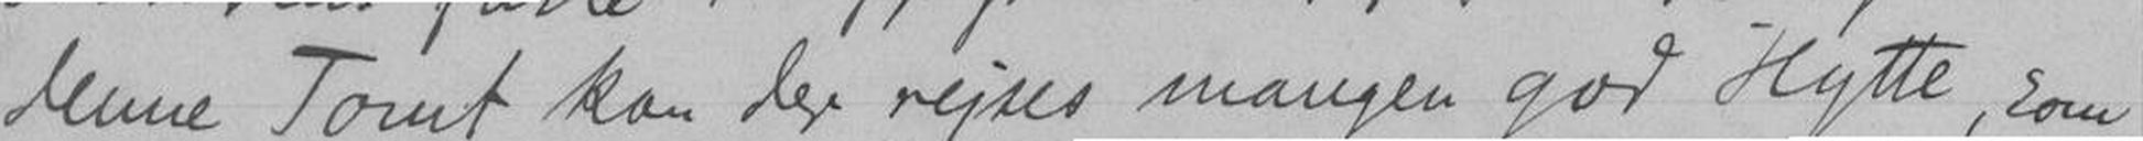denne Tomt kan der rejses mangen god Hytte, som
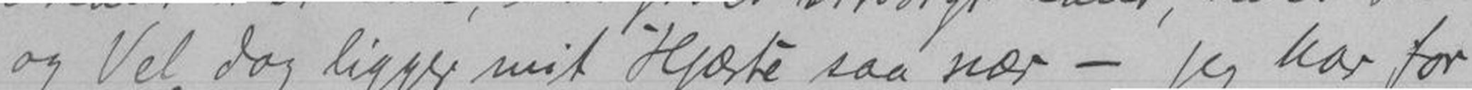og Vel dog ligger mit Hjærte saa nær - jeg har for
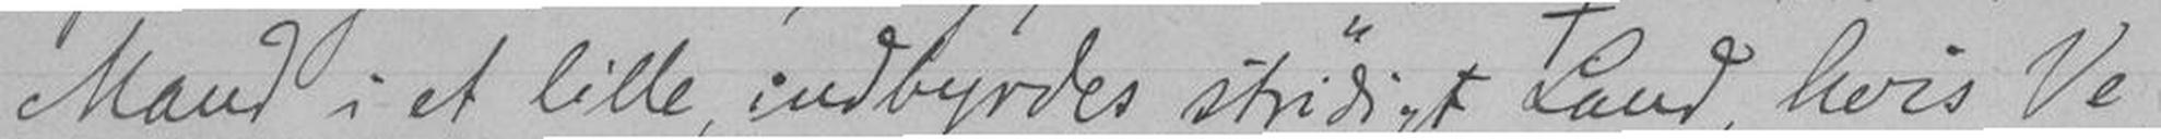Mand i et lille, indbyrdes stridigt Land, hvis Ve
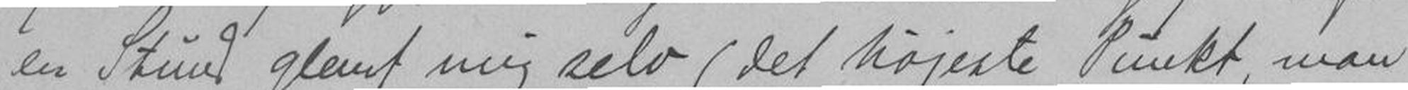en stund glemt mig selv (det højste Punkt, man
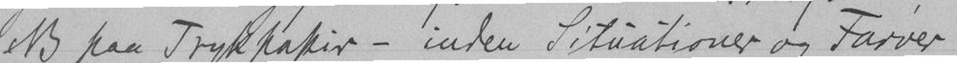NB paa Trykpapir - inden Situationer og Farver
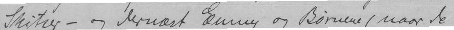Skitser - og dernæst Emmy og Børnene (naar de
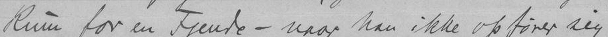Rum for en Fjende - naar han ikke opfører sig
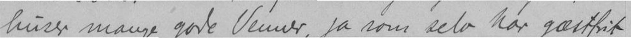huser mange gode Venner, ja som selv har gæstfrit
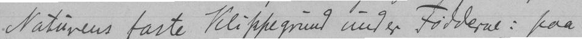Naturens faste Klippegrund under Fødderne: paa
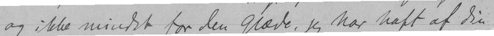og ikke mindst for den Glæde, jeg har haft af din
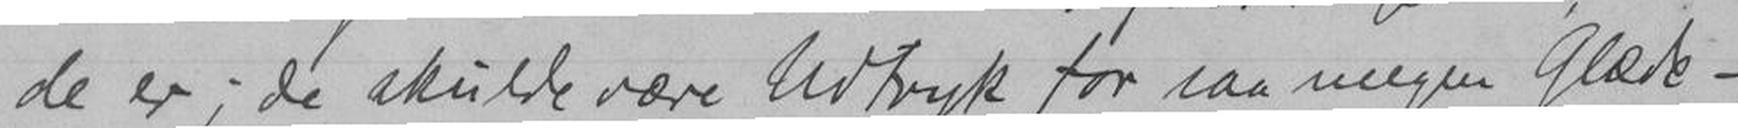de er; de skulde være Udtryk for saa megen Glæde -
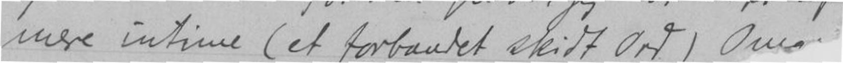mere intime (et forbandet skidt Ord). Om
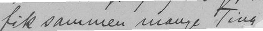fik sammen mange Ting
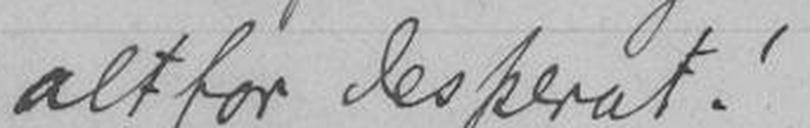altfor desperat!
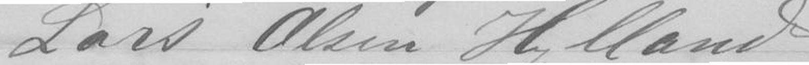Lars Olsen Hylland
